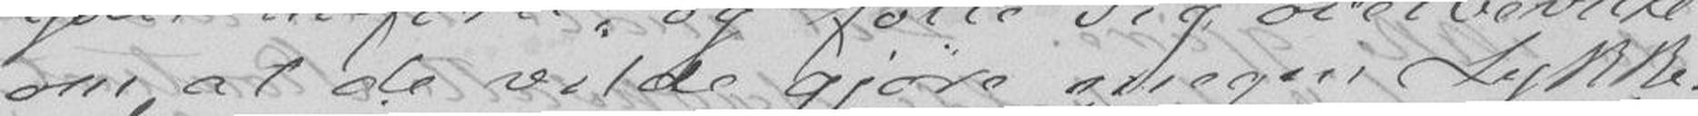om at de vilde gjøre mangen Lykke.
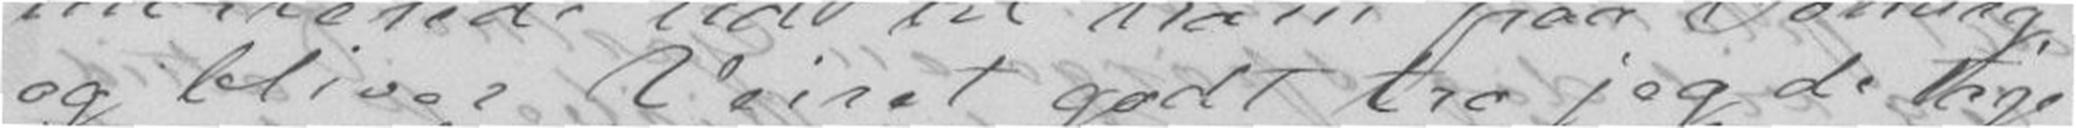og bliver Veiret godt tro jeg de tage
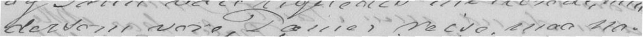dersom vore Damer reise, maa na-
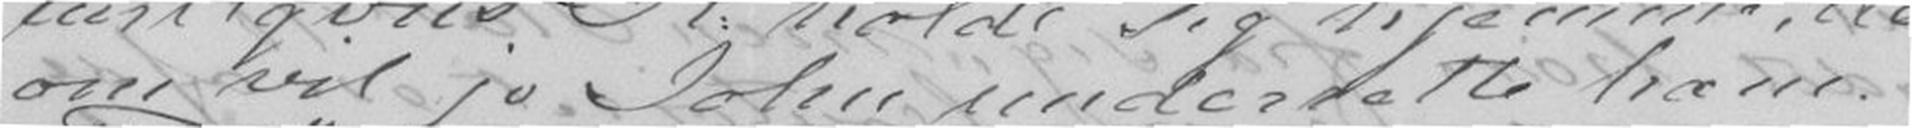om vil jo John underrette ham.
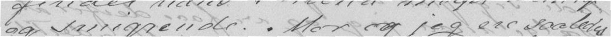og smigrende. Mor og jeg ere saaledes
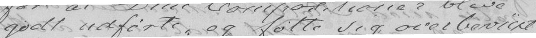godt udførte, og følte sig overbevist
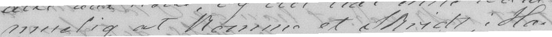muelig at komme et Skridt i Ha-
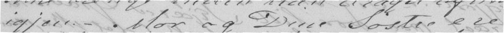igjen. - Mor og Dine Søstre ere
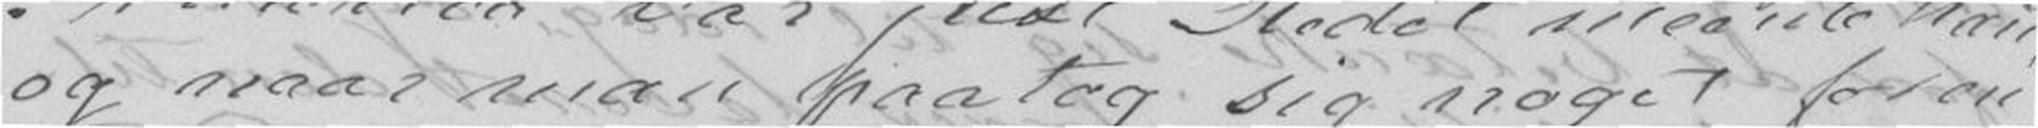og naar man paatog sig noget for en
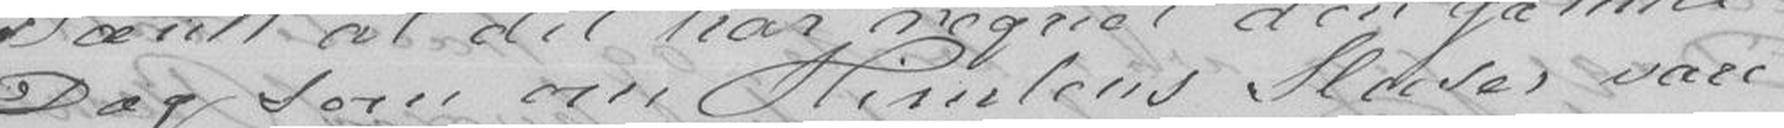Dag som om Himlens Sluser vare
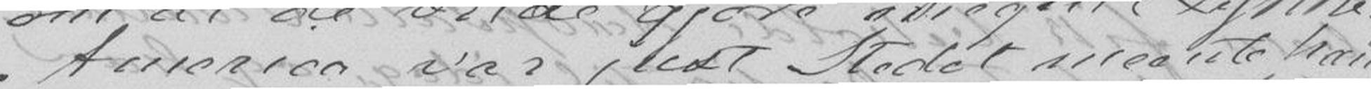America var just Stedet meente han
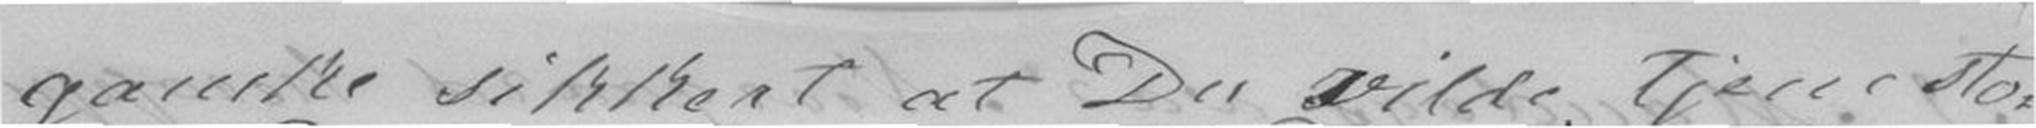ganske sikkert at Du vilde tjene sto-
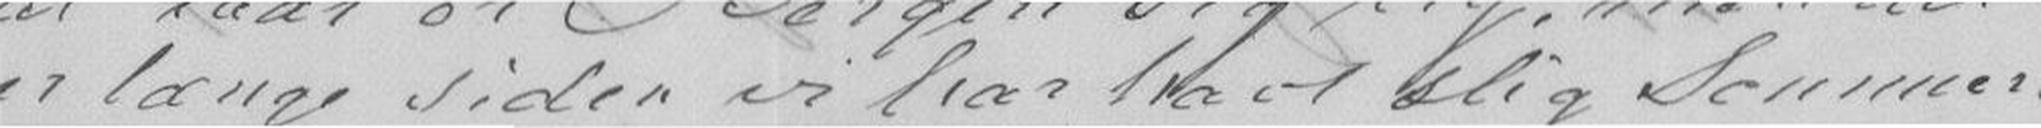er længe siden vi har havt slig Sommer.
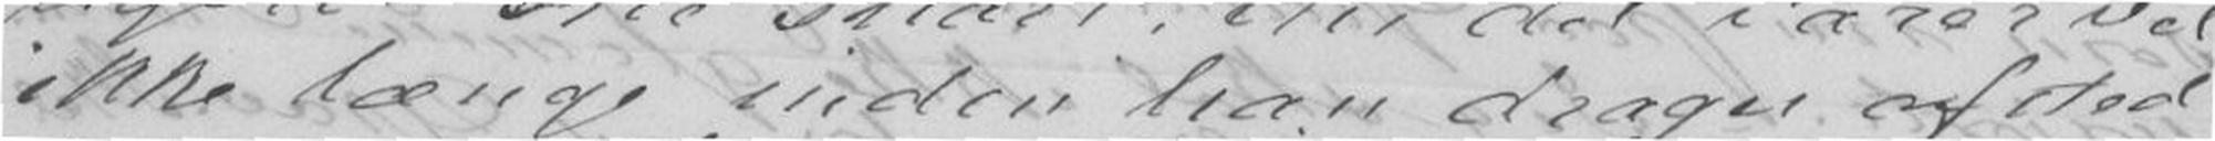ikke længe inden han drager afsted
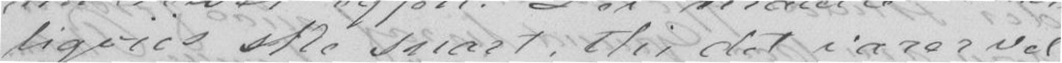ligviis ske snart, thi det varer vel
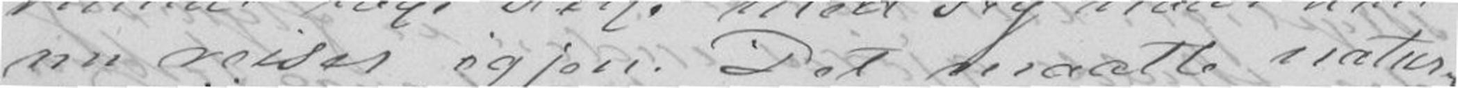nu reiser igjen. Det maatte natur-
